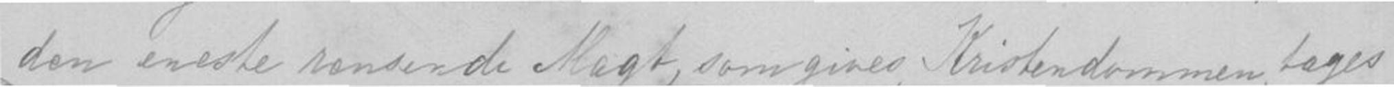den eneste rensende Magt, som gives Kristendommen, tages
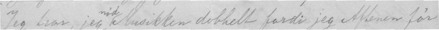Jeg tror, jeg nød Musikken dubhelt fordi jeg Aftenen før
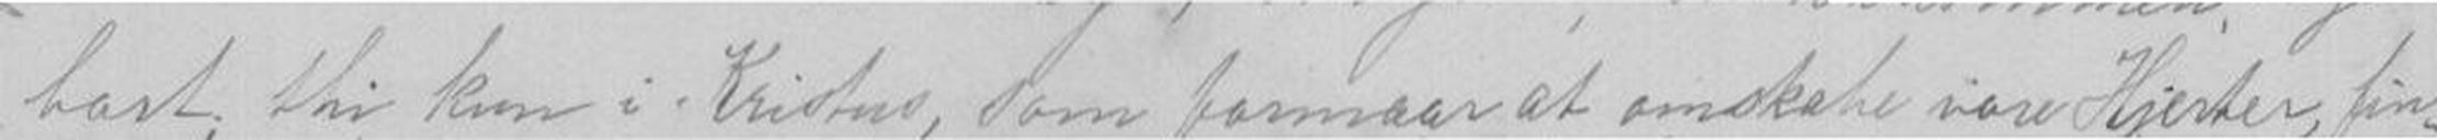bort thi kan i Kristus, som framraar at omskabe vore hjerter
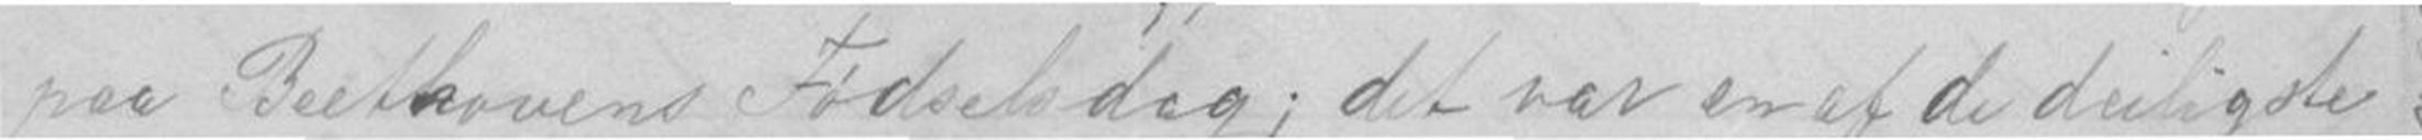paa Beethovens Fødselsdag; det var en af de deiligste
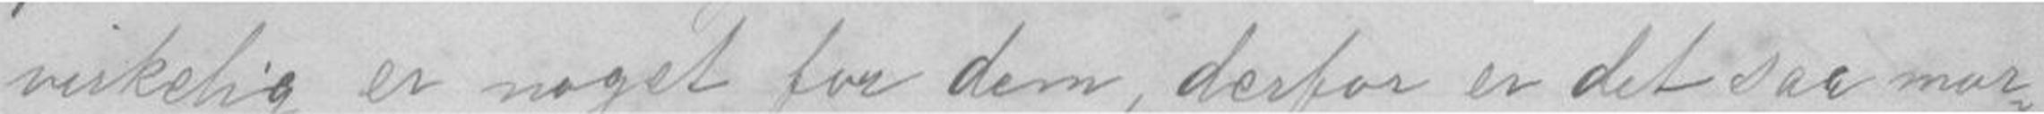virkelig er noget for dem, derfor er det saa mor-
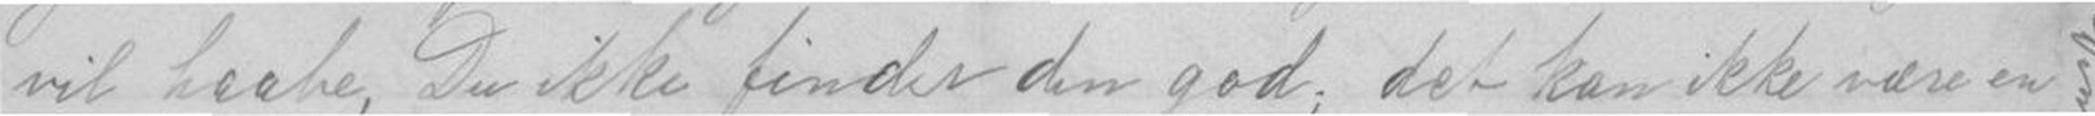vil haabe, Du ikke finder den god; det kan ikke være en
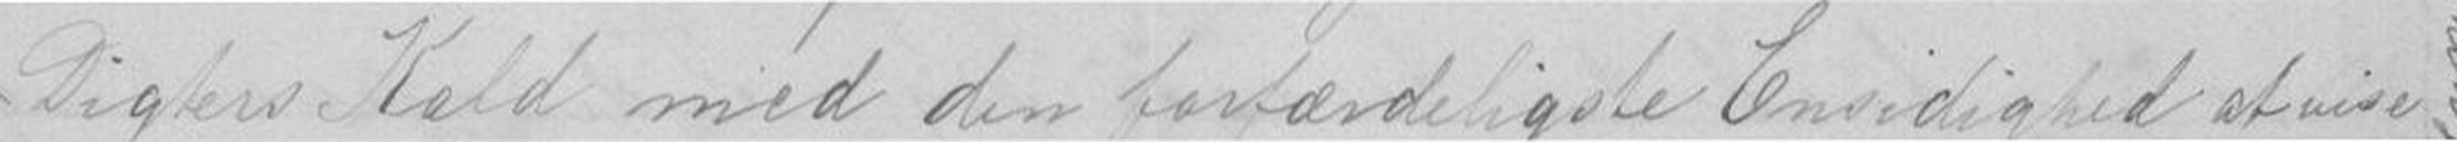Digters Keld med den forfærdeligste Ensidighed at vise
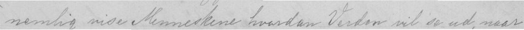nemlig vise Menneskene hvordan Verden vil se ud, naar
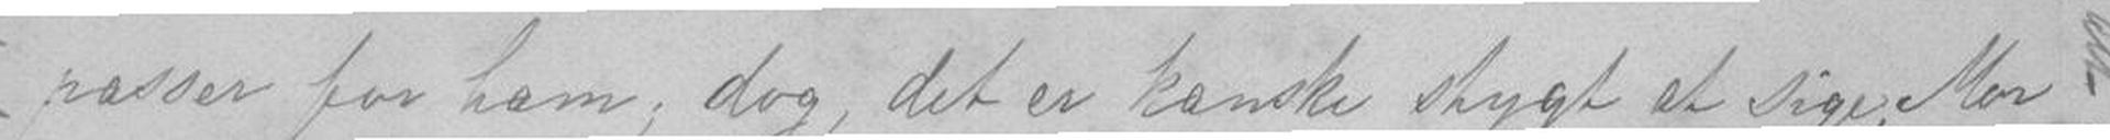passer for ham; dog, det er kanske stygt at sige, mor
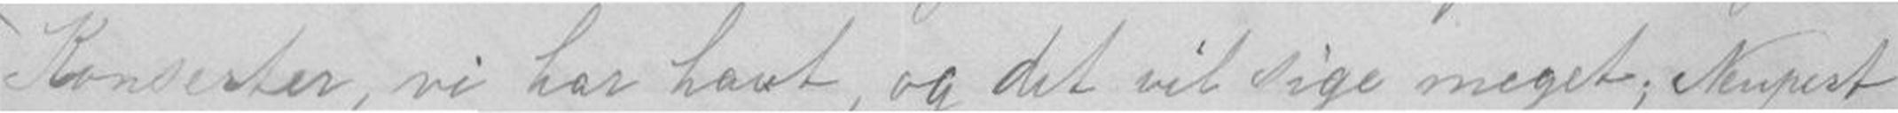Konserter, vi har havt, og det vil sige meget; Neupert
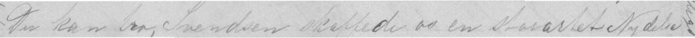Du kan tro, Svendsen skaffede og en storartet Nydelse
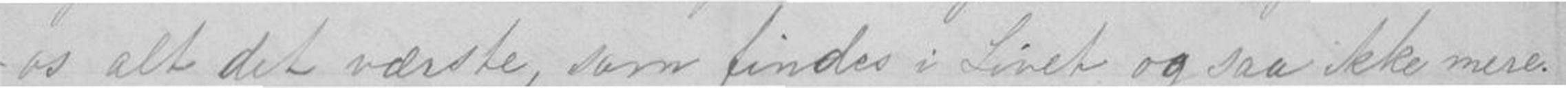os alt det værste, som findes i Livet og saa ikke mere.
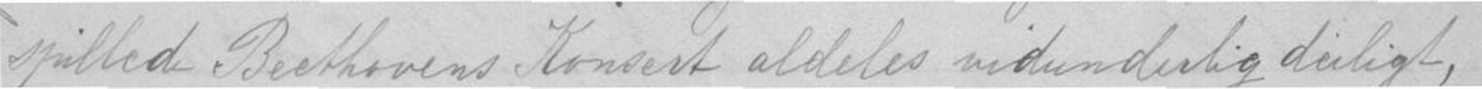spillede Beethovens Konsert aldeles vidunderlig deiligt,
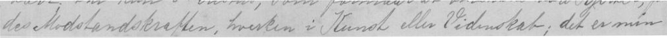fin=desModstandskraften, hverken i Kunst eller Videnskab;det er min
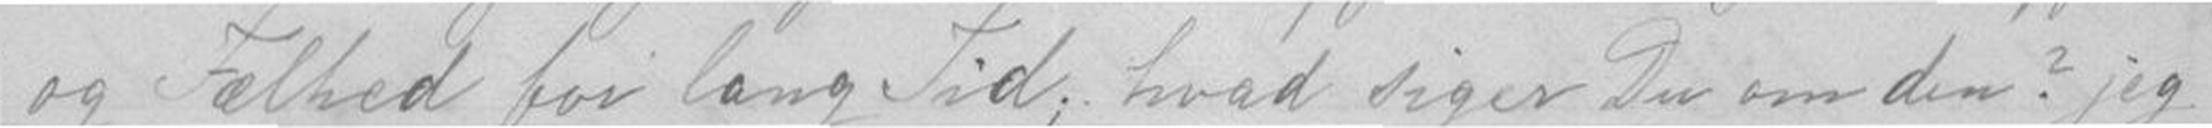og Fælhed for lang Tid; hvad siger Du om den? jeg
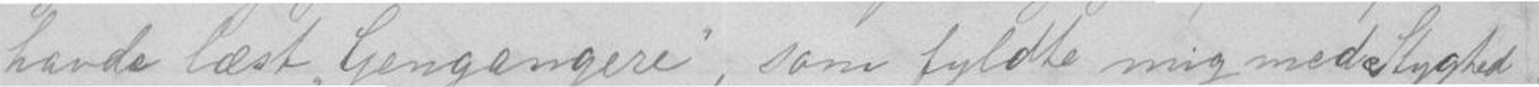havde læst "Gengangere" som fyldte mi med Plyghed
